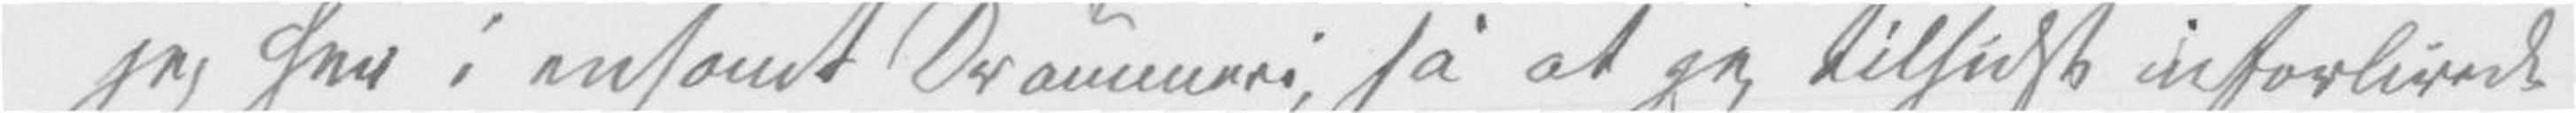jeg her i ensomt Drømmeri, så at jeg tilsidst inforlivede
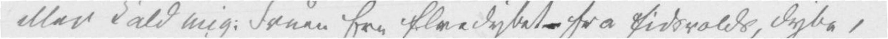eller kald mig: «Fruen fra Elvedybet[»] – fra Eidsvolds, dybe,
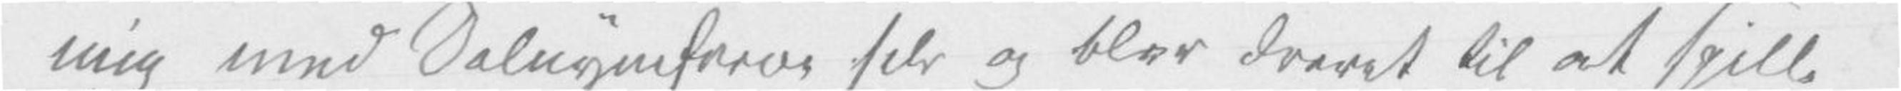mig med Dalnymferne selv og blev drevet til at spille
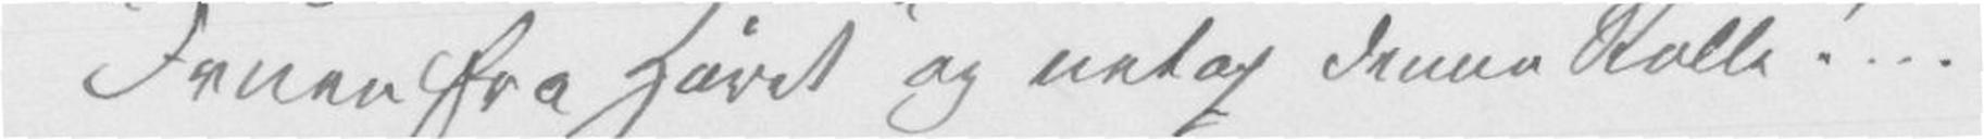«Fruen fra Havet» og netop denne Rolle! ...
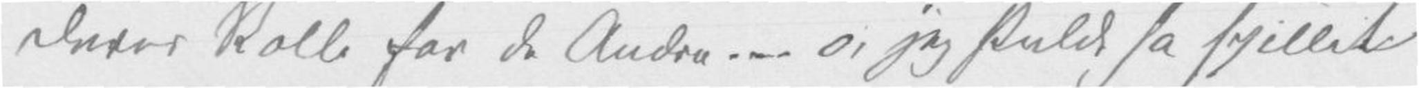deres Rolle for de Andre ... o, jeg skulde ha spillet
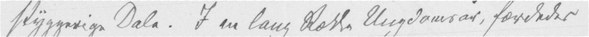skyggerige Dale. I en lang Række Ungdomsår, færdedes
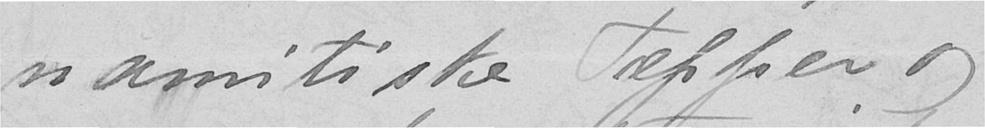namitiske Tæpper og
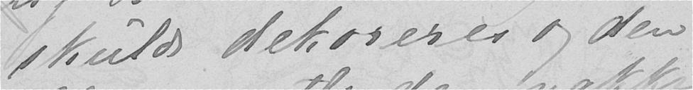skulde dekoreres og den
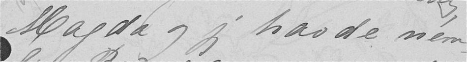Magda og jeg havde nem-
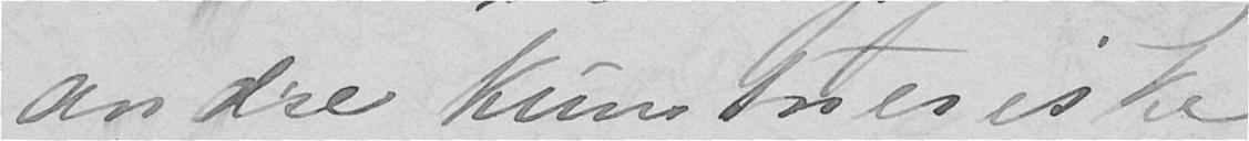andre kunstneriske
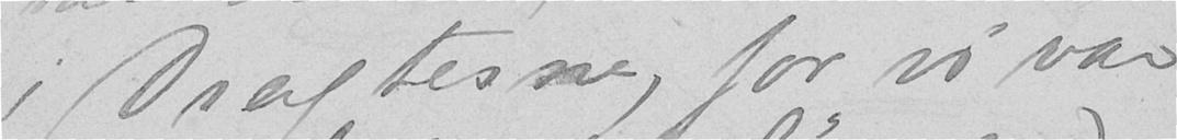i Dragterne, for vi var
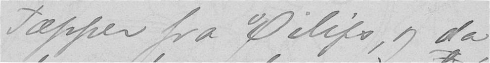Tæpper fra Eilifs, og da
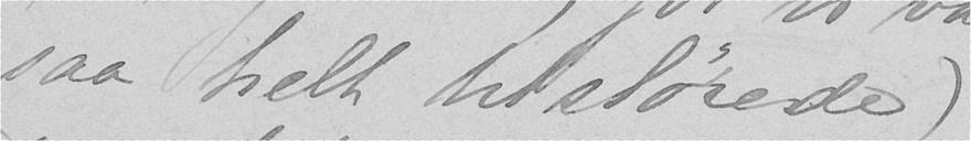saa helt tilslørede)
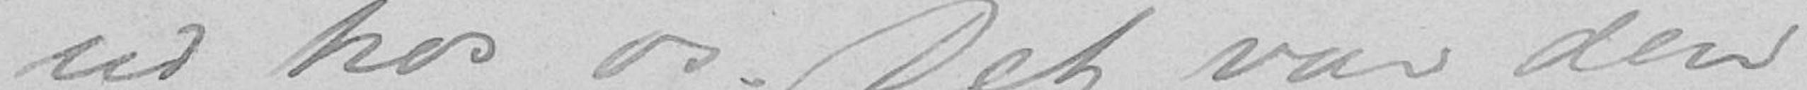ud hos os. Det var den
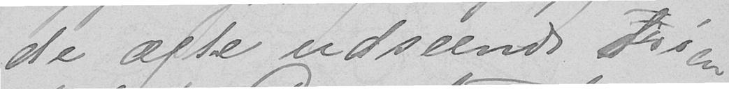de ægte udseende orien-
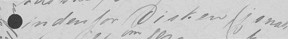indenfor Disken, (jeg snak-
